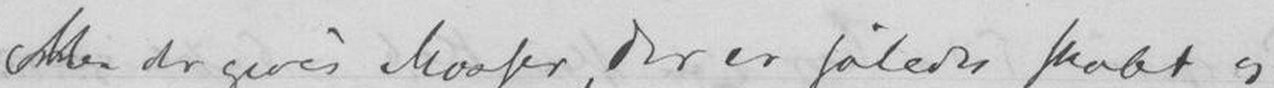Men der gives Masser, der er således skabt og
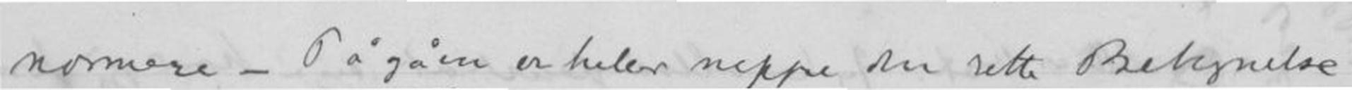nærmere - Pågåen er heler neppe den rette Betegnelse
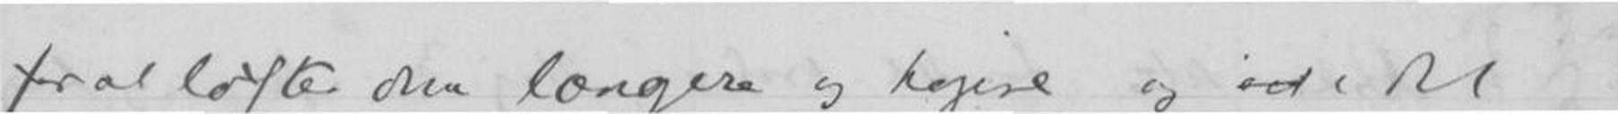for at løfte den længere og højere og id i det
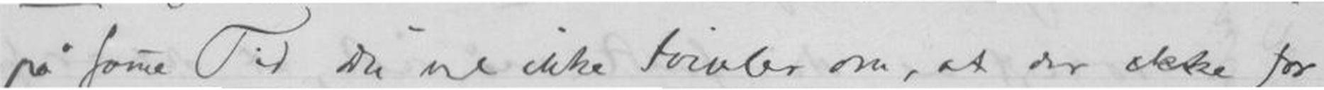på same Tid Du vil ikke tvivles om, at der ikke for
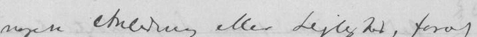neste Anledning eller Lejlighed, forat
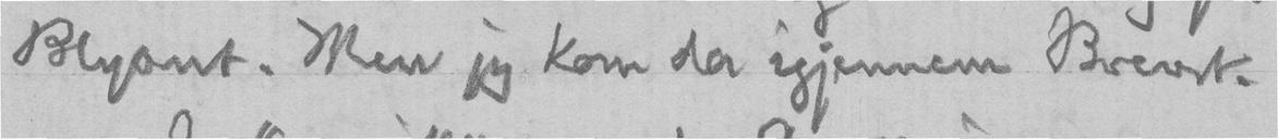Blyant. Men jeg kom da igjennem Brevet.
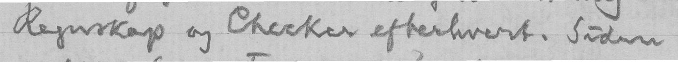Regnskap og Checker efterhvert. Siden
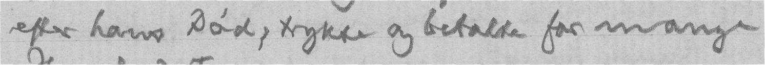efter hans Død, trykte og betalte for mange
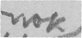nok
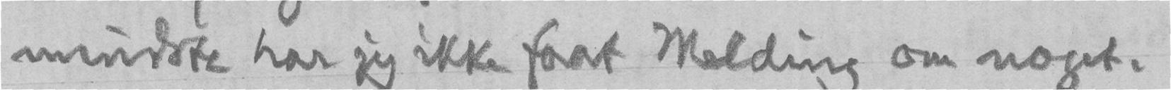mindste har jeg ikke faat Melding om noget.
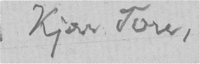Kjære Tore,
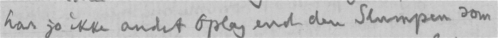har jo ikke andet Oplag end den Slumpen som
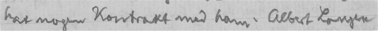hat nogen Kontrakt med ham. Albert Langen
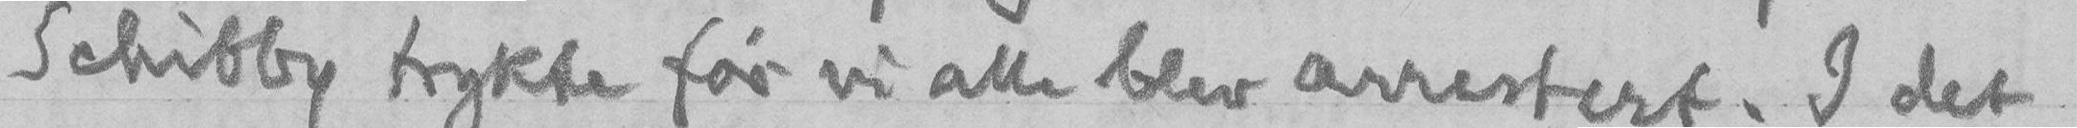Schibby trykte før vi alle blev arrestert. I det
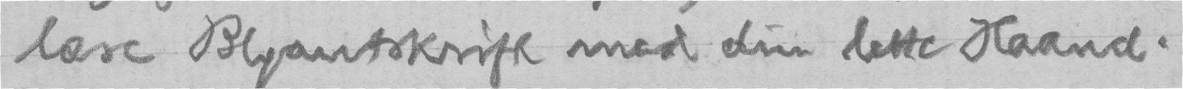læse Blyantskrift med din lette Haand.
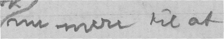nu mere til at
